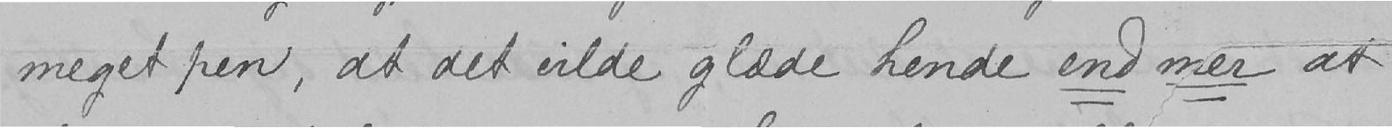meget pen, at det vilde glæde hende end mer at
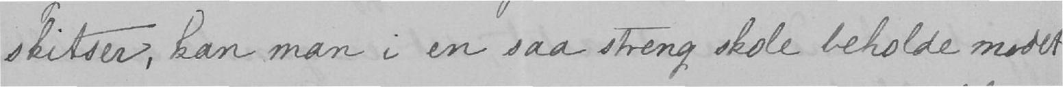skitser, kan man i en saa streng skole beholde modet
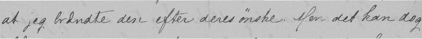at jeg brændte den efter deres ønske. Men det kan dog
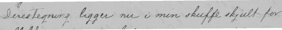Deres tegning ligger nu i min skuffe skjult for
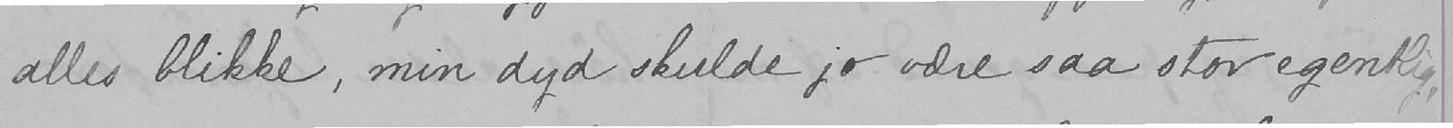alles blikke, min dyd skulde jo være saa stor egentlig,
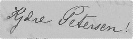Kjære Petersen!
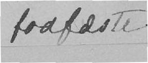fodfæste
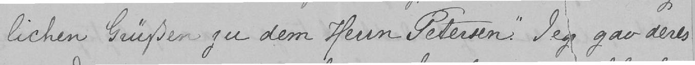lichen Grüssen zu dem Herrn Petersen». Jeg gav deres
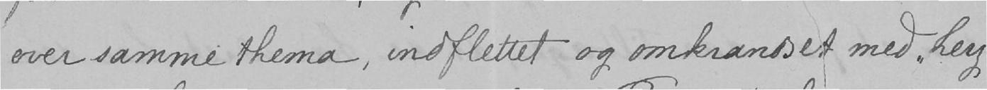over samme thema, indflettet og omkrandset med «herz-
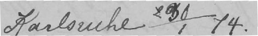Karlsruhe 230/1 74
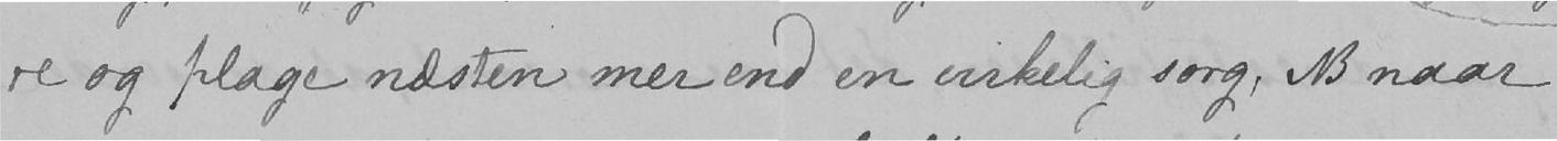re og plage næsten mer end en virkelig sorg, NB naar
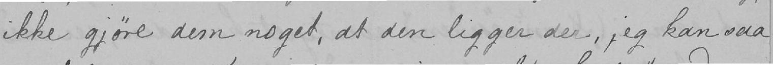ikke gjøre dem noget, at den ligger der, jeg kan saa
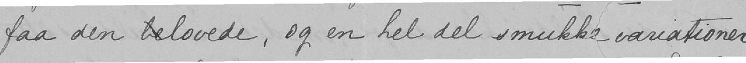faa den belovede, og en hel del smukke variationer
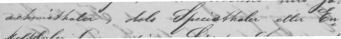achimsthaler, dels Speciethaler eller En-
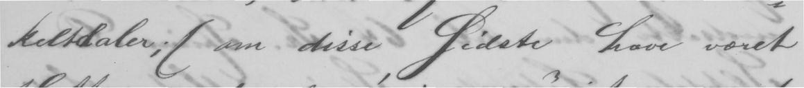keltdaler; (om disse Sidste have været
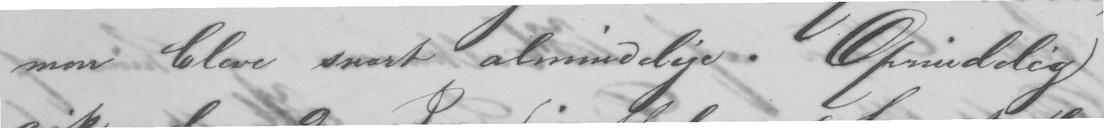men bleve snart almindelige. Oprindelig
